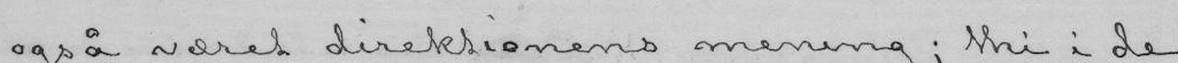også været direktionens mening; thi i de
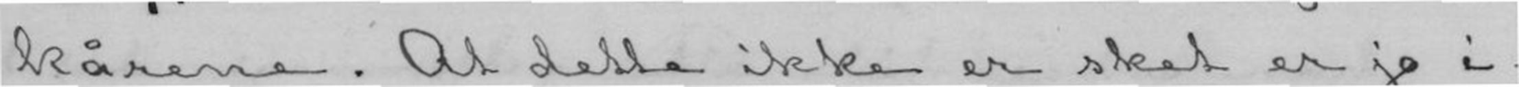kårene. At dette ikke er sket er jo i-
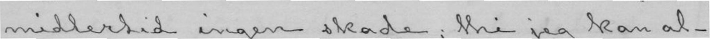midlertid ingen skade; thi jeg kan al-
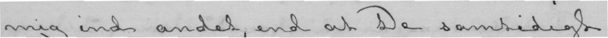mig ind andet, end at De samtidigt
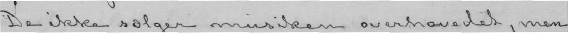De ikke sælger musiken overhovedet, men
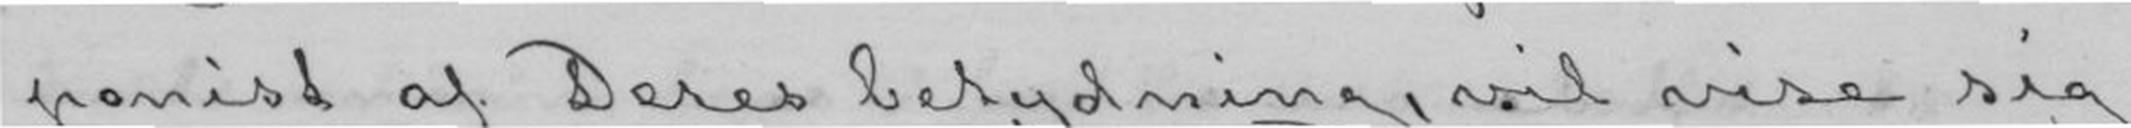ponist af Deres betydning vil vise sig
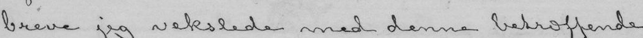breve jeg vekslede med denne betræffende
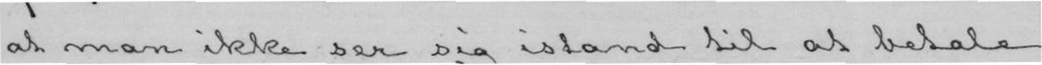at man ikke ser sig istand til at betale
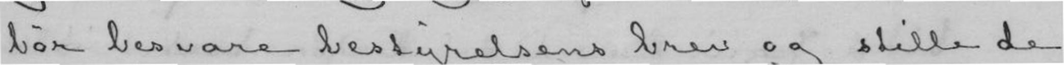bør besvare bestyrelsens brev og stille de
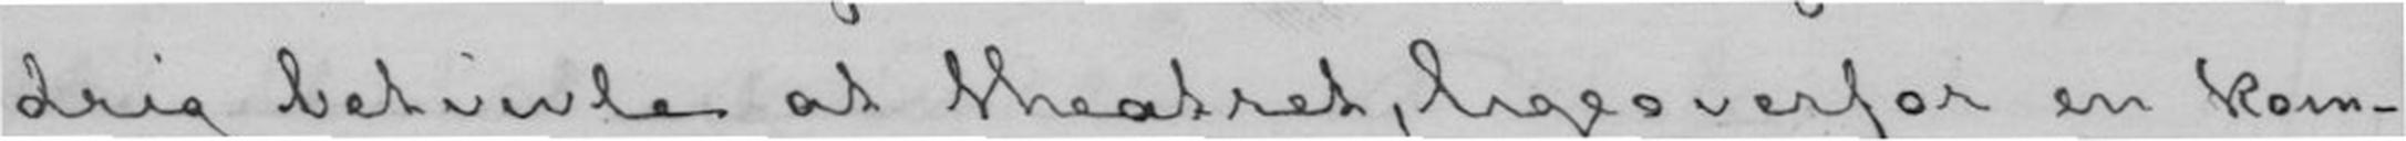drig betvivle at theatret, ligeoverfor en kom-
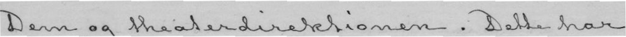Dem og theaterdirektionen. Dette har
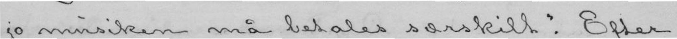jo musiken må betales særskilt". Efter
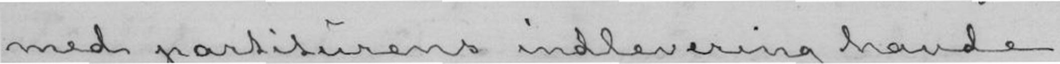med partiturens indlevering havde
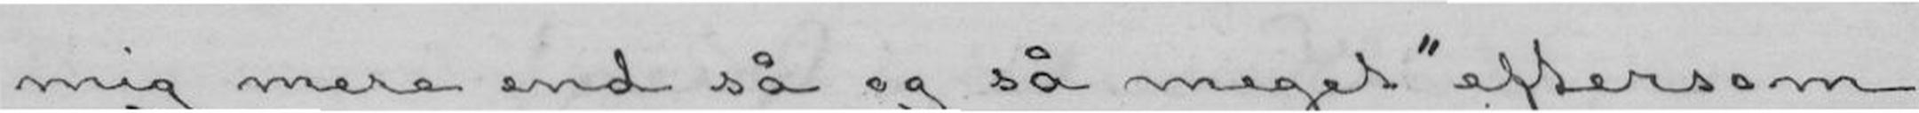mig mere end så og så meget "eftersom
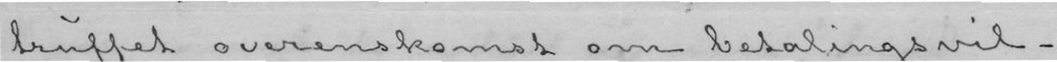truffet overenskomst som betalingsvil-
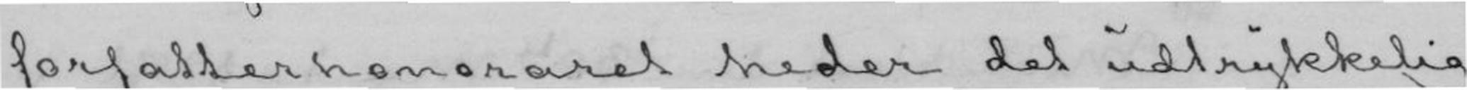forfatterhonoraret heder det udtrykkelig
